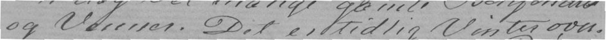og Venner. Det er tidlig Vinter over-
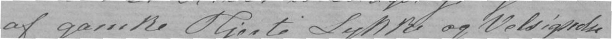af ganske Hjerte Lykke og Velsignelse
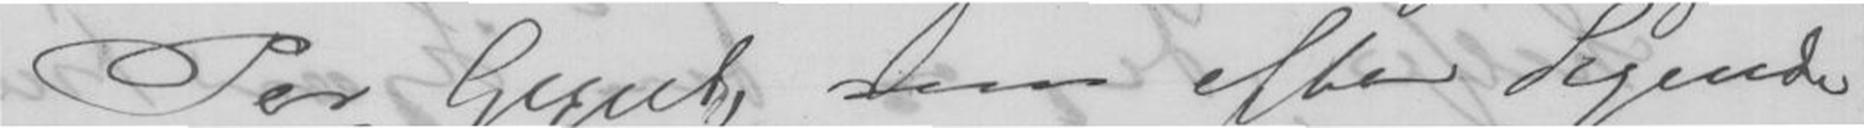Per Gynt, som efter Sigende
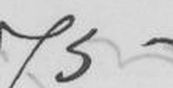75
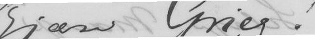Kjære Grieg!
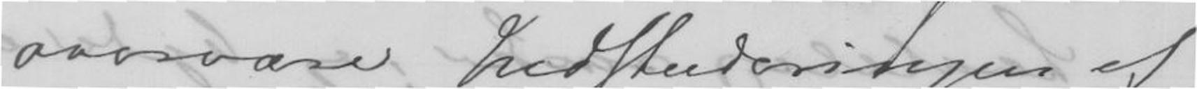overvære Indstuderingen af
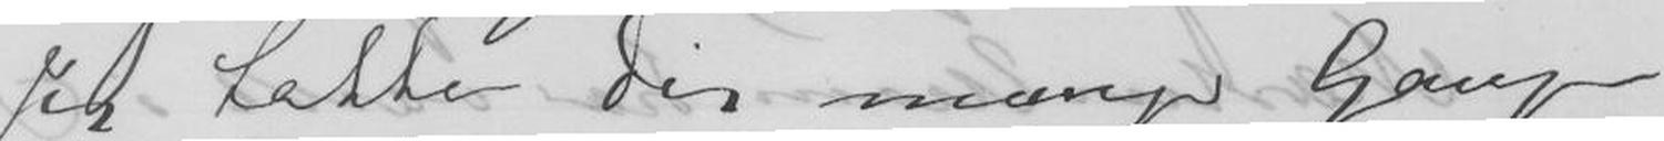Jeg takker Dig mange Gange
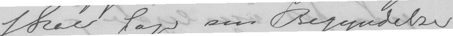skal tage sin Begyndelse
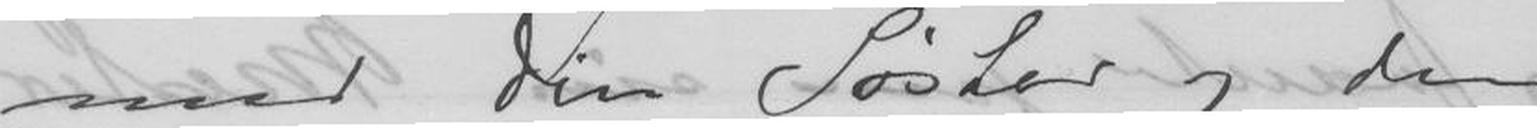med din Søster og den
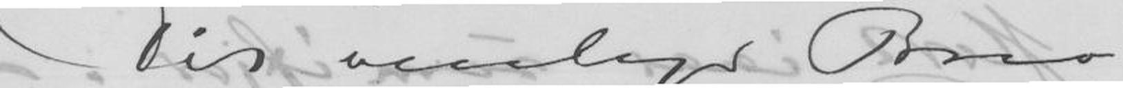Dit venlige Brev
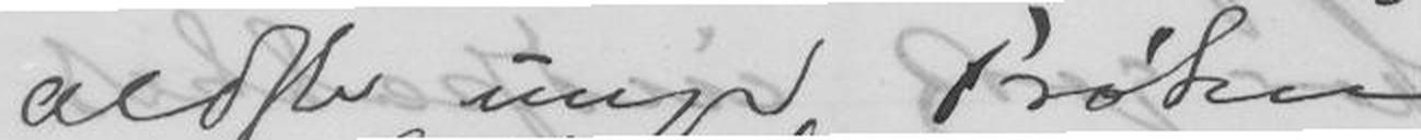ældste unge Frøken.
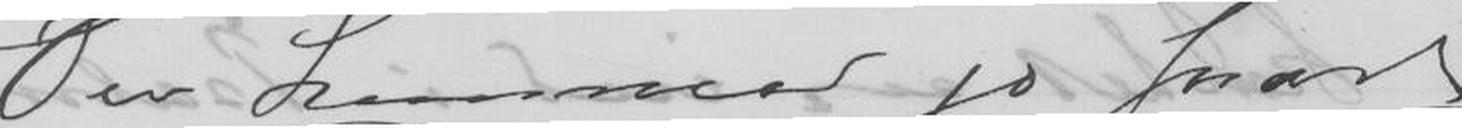Du kommer jo snart
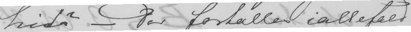hid? - Det fortælles iallefald
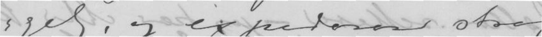get, og expederer strax
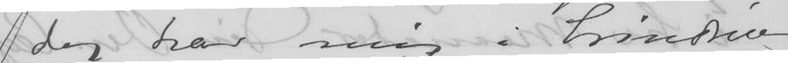dog har mig i Erindring.
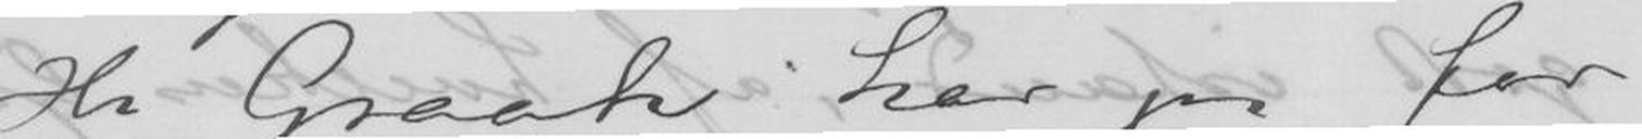Hr Graah har jeg for
